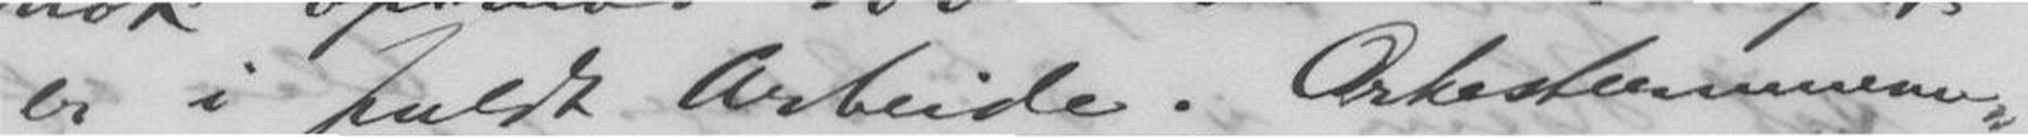er i fuldt Arbeide. Orkesternumme-
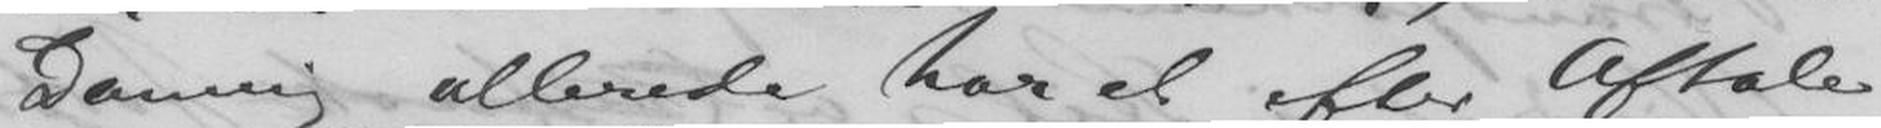Danning allerede har et efter Aftale
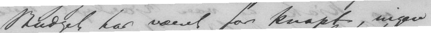Budget har været for Knapt, ingen
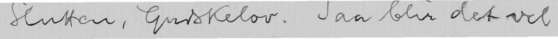Slutten, Gudskelov. Saa blir det vel
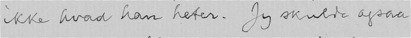ikke hvad han heter. Jeg skulde ogsaa
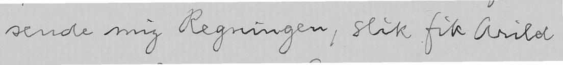sende mig Regningen, slik fik Arild
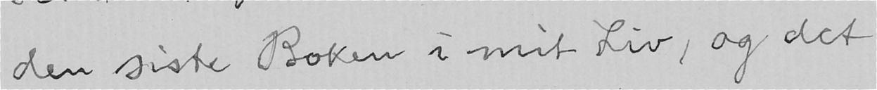den siste Boken i mit Liv, og det
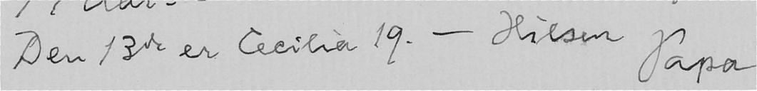Den 13de er Cecilia 19. - Hilsen Papa
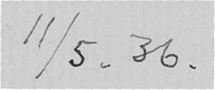11/5. 36.
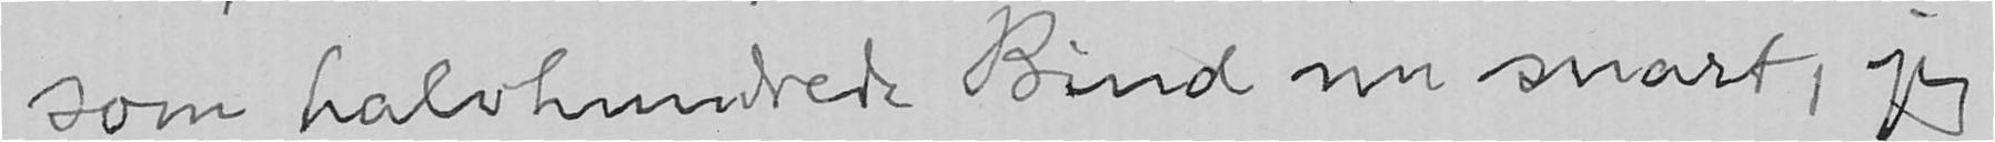som halvhundrede Bind nu snart, jeg
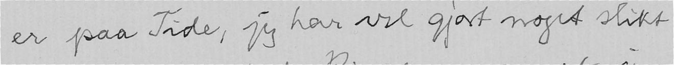er paa Tide, jeg har vel gjort noget slikt
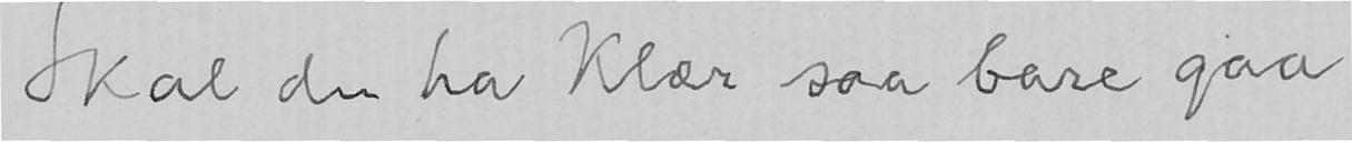Skal du ha Klær saa bare gaa
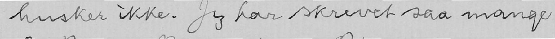husker ikke. Jeg har skrevet saa mange
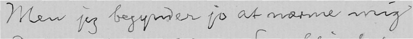Men jeg begynder jo at nærme mig
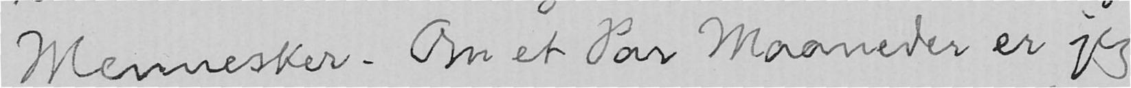Mennesker. Om et Par Maaneder er jeg
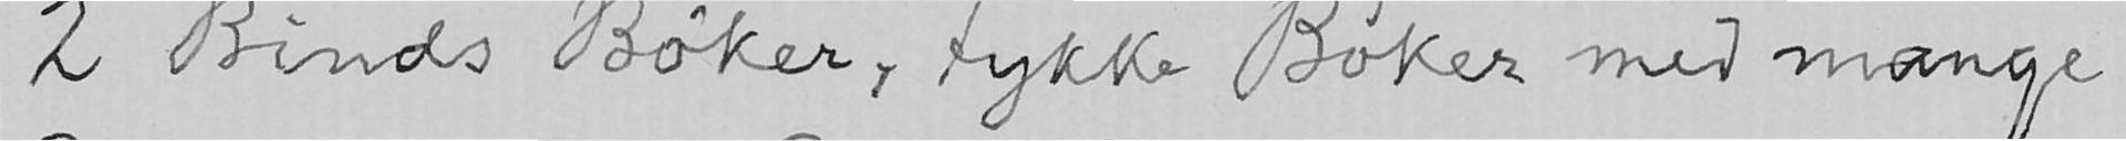2 Binds Bøker, tykke Bøker med mange
